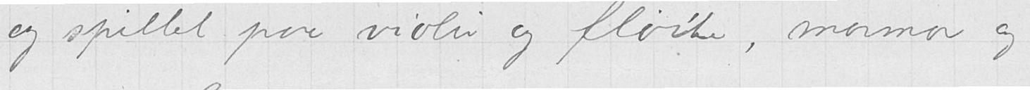og spillet paa violin og fløite, mormor og
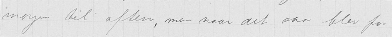morgen til aften, men naar det saa blev for
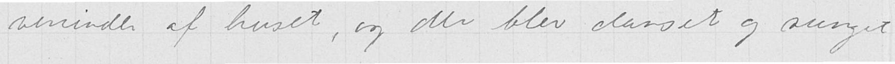veninder af huset, og der blev danset og sunget
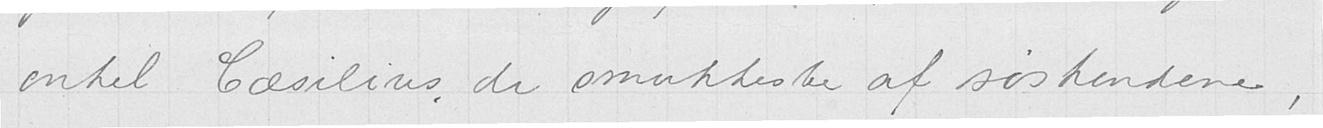onkel Cæsilius, de smukkeste af søskendene,
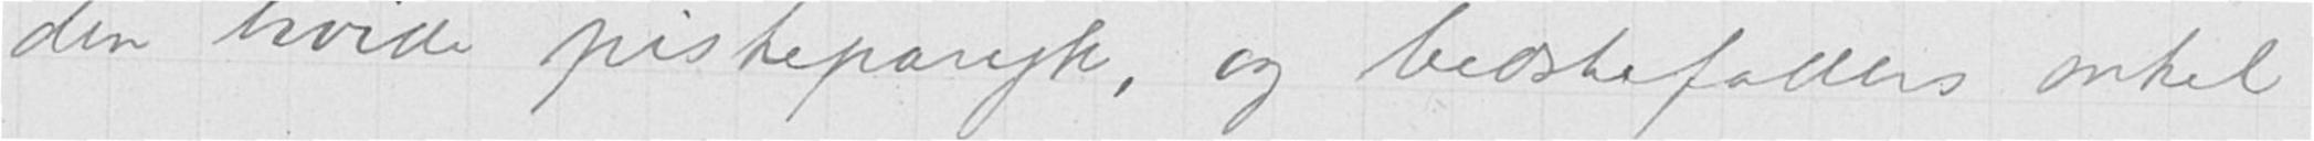den hvide pisteparyk, og bedstefaders onkel
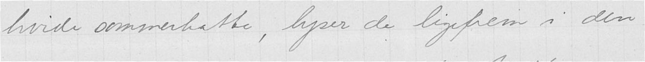hvide sommerhatte, lyser de ligefrem i den
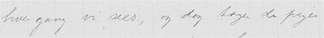hver gang vi sees, og dog tager de piger
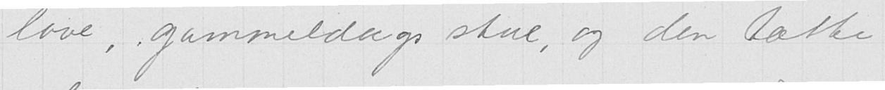lave, gammeldags stue, og den tætte
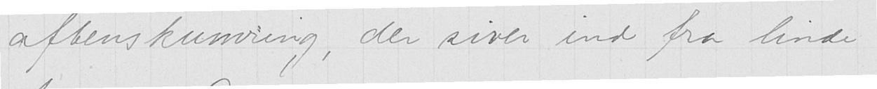aftenskumring, der siver ind fra linde-
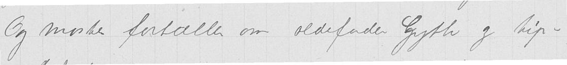Og moster fortæller om oldefader Gyth og tip-
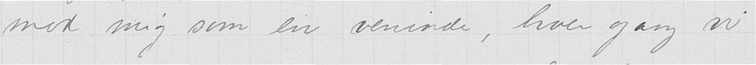mod mig som en veninde, hver gang vi
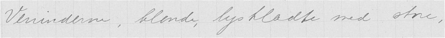Veninderne, blonde, lysklædte med store,
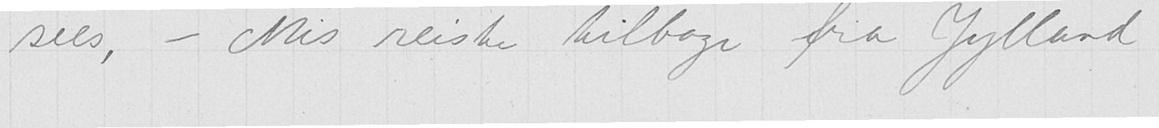sees, - Mis reiste tilbage fra Jylland
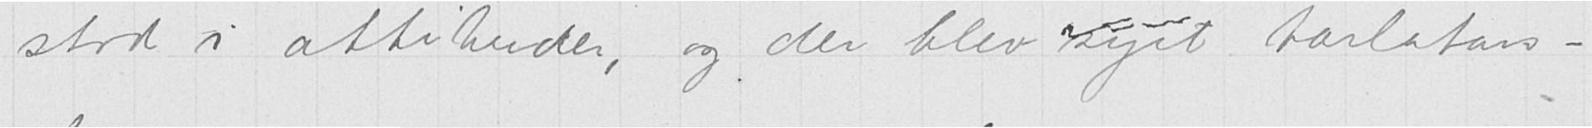stod i attituder, og der blev syet tarlatans-
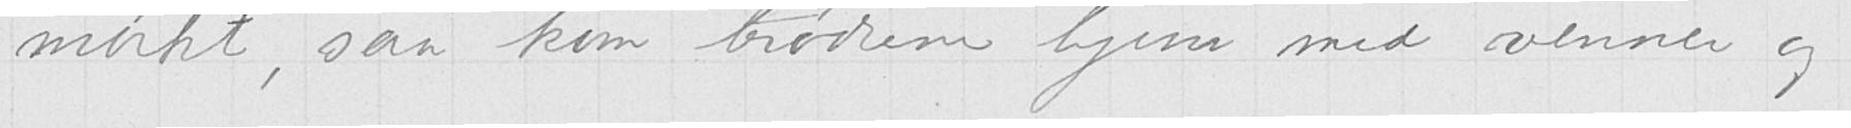mørkt, saa kom brødrene hjem med venner og
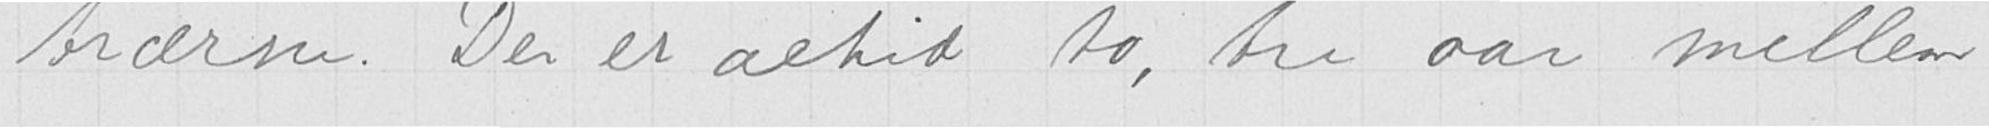trærne. Der er altid to, tre aar mellem
